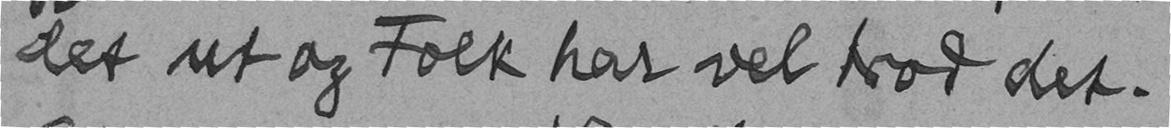det ut og Folk har vel trod det.
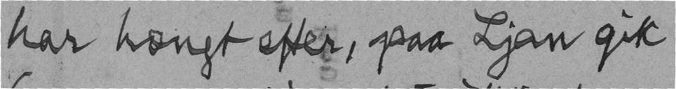har hængt efter, paa Ljan gik
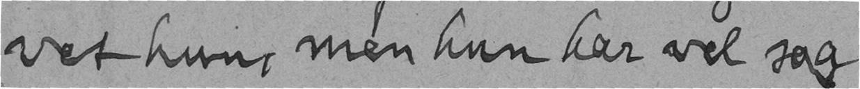vet hun, men hun har vel saa
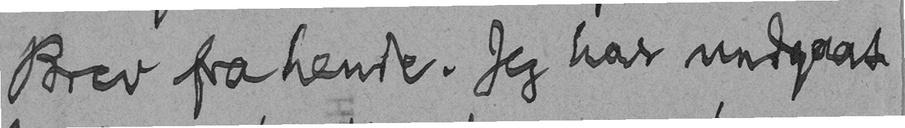Brev fra hende. Jeg har undgaat
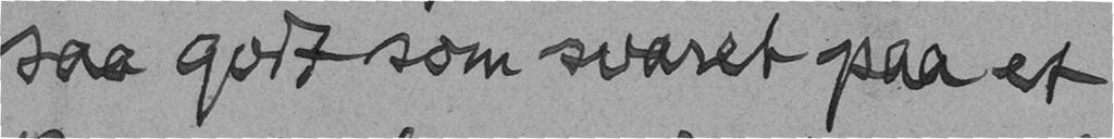saa godt som svaret paa et
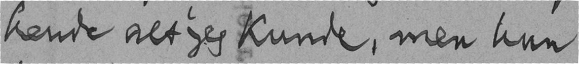hende alt jeg kunde, men hun
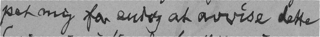pet mig for endog at avvise dette
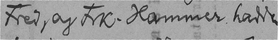Fred, og Frk. Hammer hadde
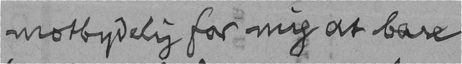motbydelig for mig at bare
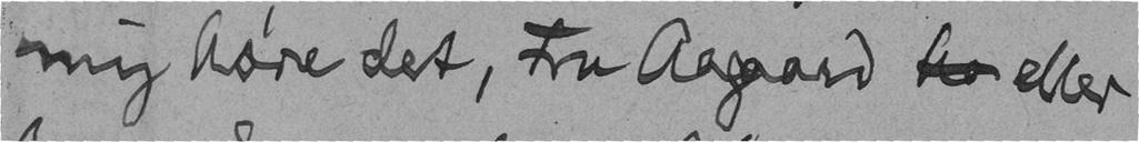mig høre det, Fru Aagard he eller
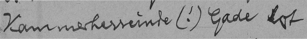Kammerherreinde (!) Gade lot
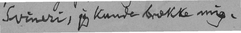Svineri, jeg kunde brække mig.
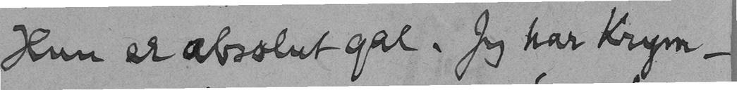Hun er absolut gal. Jeg har krym-
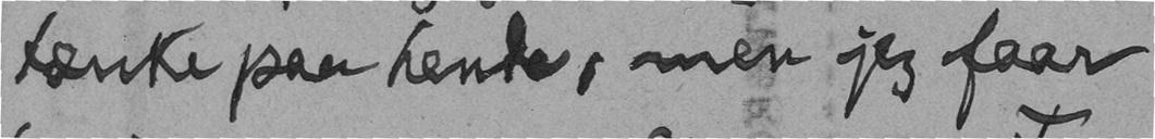tænke paa hende, men jeg faar
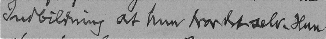Indbildning at hun tror det selv. Hun
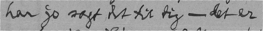har jo sagt det til dig - det er
